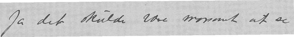Ja det skulde være morsomt at se
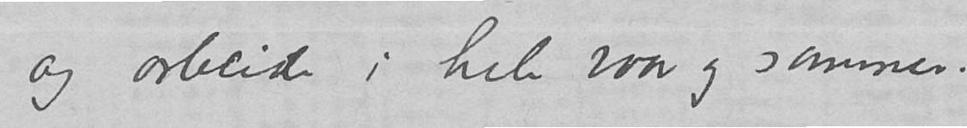og arbeide i hele vaar og sommer.
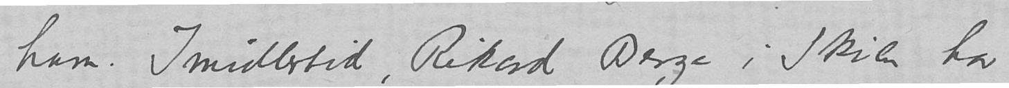ham. Imidlertid, Rikard Berge i Skien har
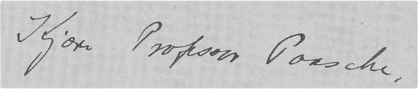Kjære Professor Paasche,
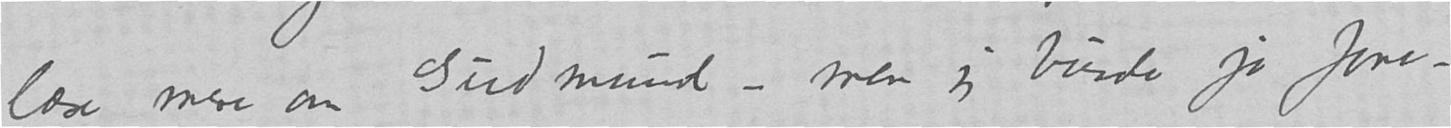læse mere om Gudmund – men jeg burde jo fore-
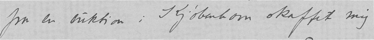fra en auktion i Kjøbenhavn skaffet mig
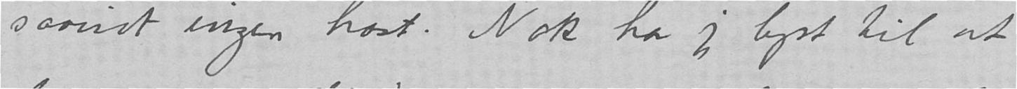saavidt ingen hast. Nok har jeg lyst til at
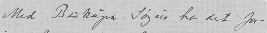Med Biskupa Sögur har det for-
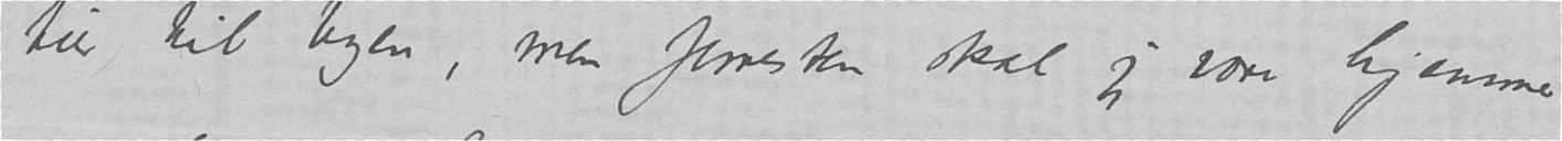tur til byen, men forresten skal jeg være hjemme
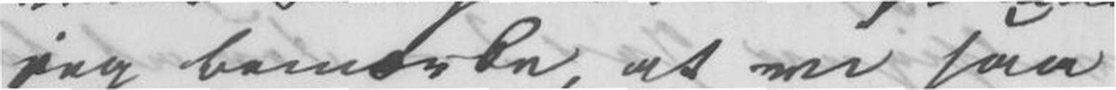jeg bemærke, at en saa
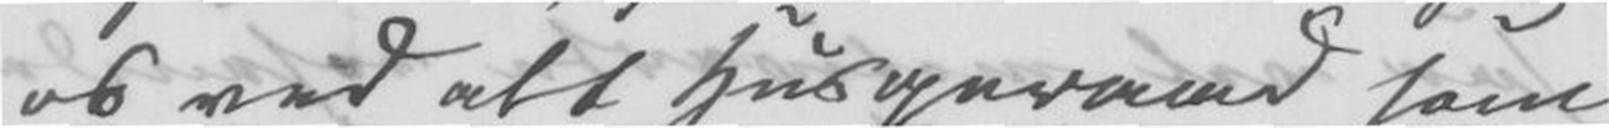os ved ett husgeraad som
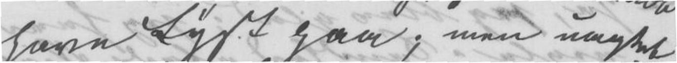have Lyst paa; men uagtet
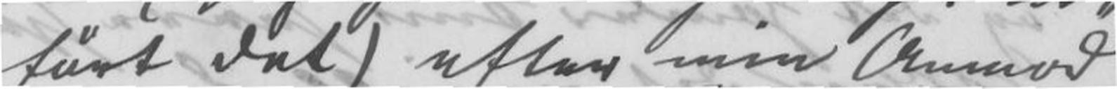ført det) efter min Anmod
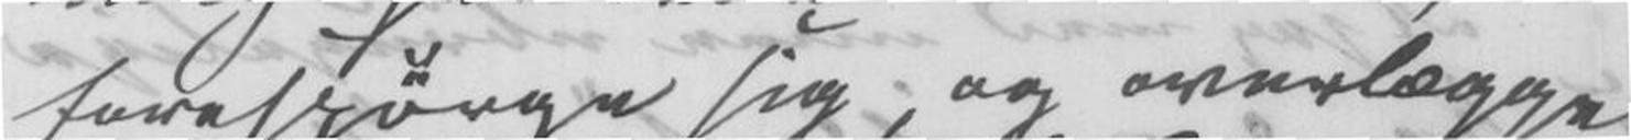forespørge sig, og overlægge
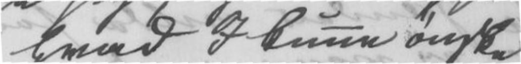hvad I kunne ønske
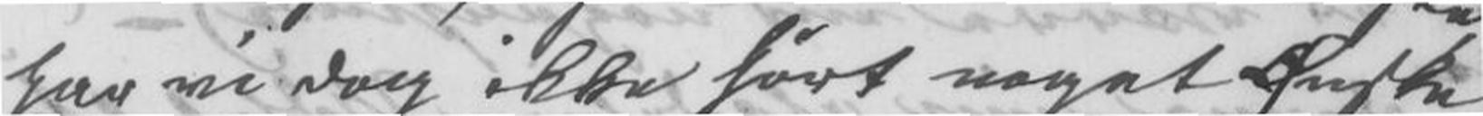har vi dog ikke hørt noget Ønske
